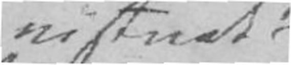vistnok
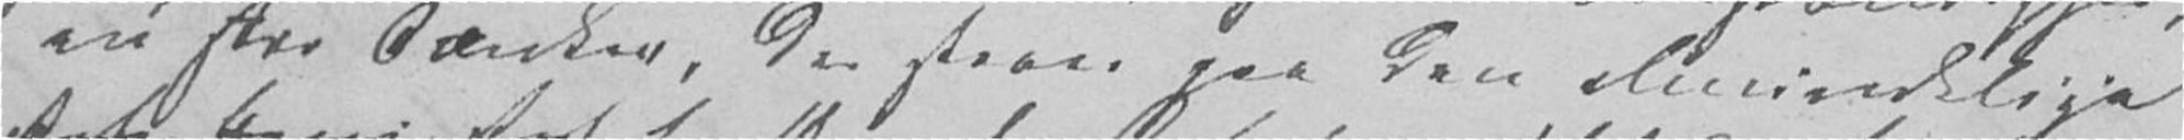en stor Tænker, der staaer paa den almindelige
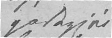godtgjør
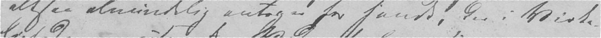altsaa almindelig antager for sandt, der i Virke-
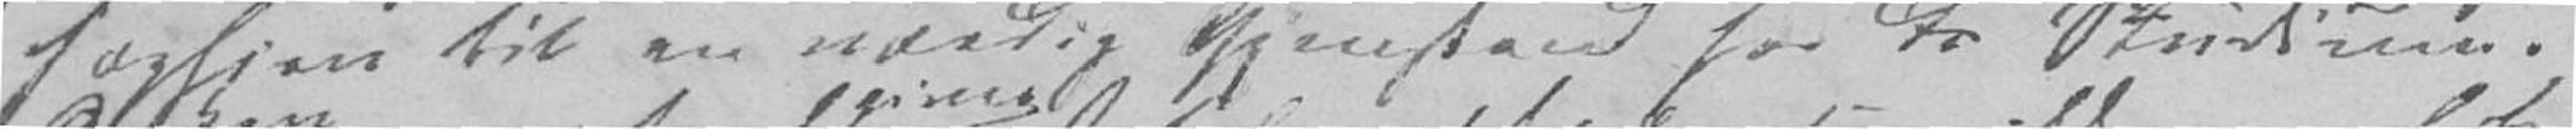sophien til en værdig Gjenstand for de Studium.
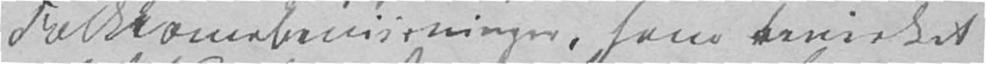Folkeoverbeviisninger, som bevirket
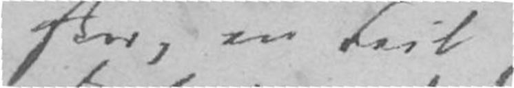ster, en Feil
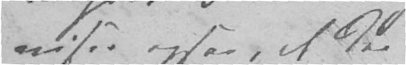viser ogsaa, at der
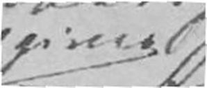gives
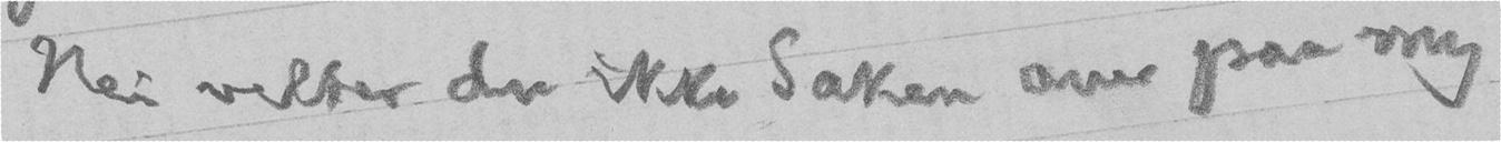Nei velter du ikke Saken over paa mig
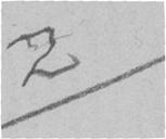2
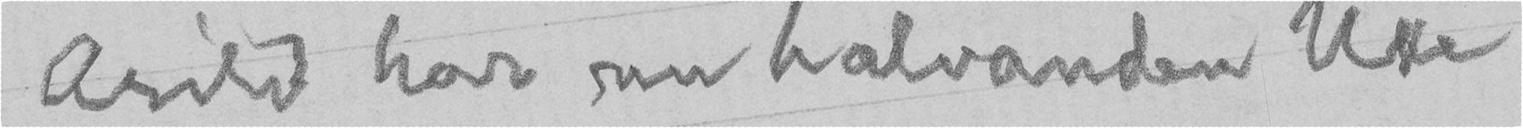Arild har nu halvanden Uke
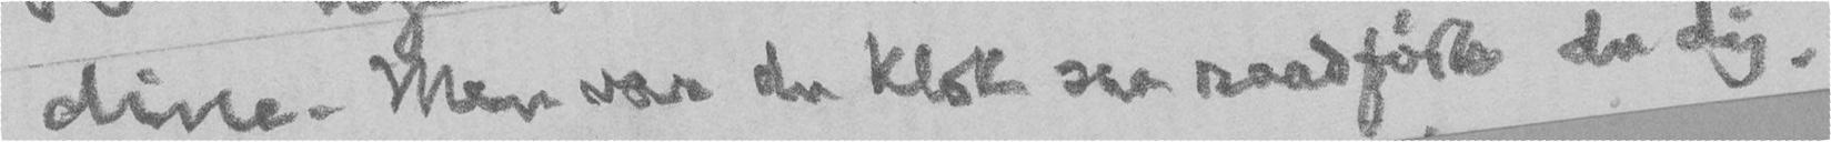dine. Men var du klok saa raadførte du dig.
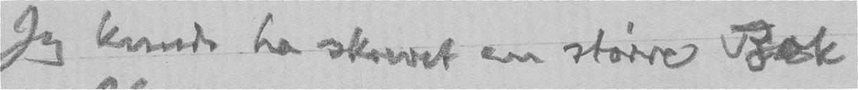Jeg kunde ha skrevet en større Bok
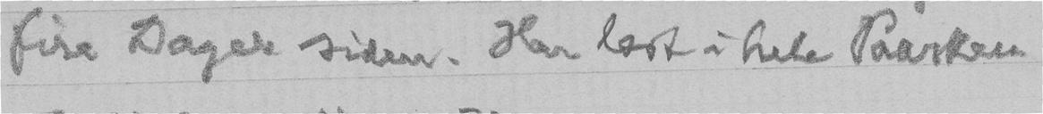fire Dager siden. Har læst i hele Paasken
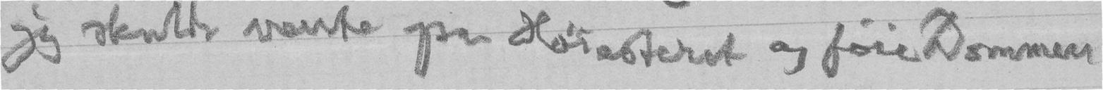jeg skulde vente paa Høiesteret og føie Dommen
